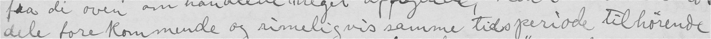dele forekommende og rimeligvis samme tidsperiode tilhørende
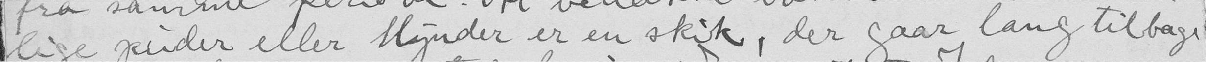lige puder eller Hynder er en skik, der gaar lang tilbage
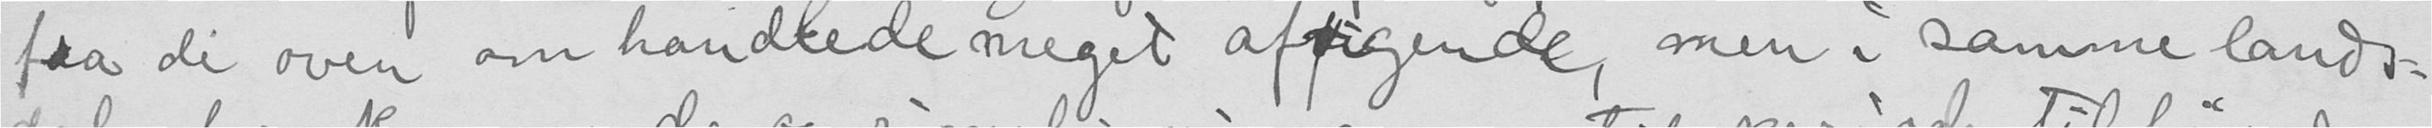fra di oven om handlede meget aftigende men i samme lands-
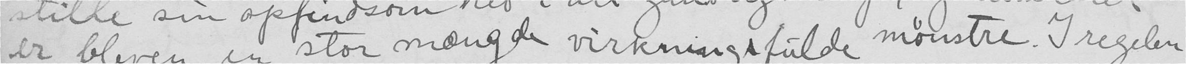er bleven en stor mængde virkningsfulde mønstre. I regelen
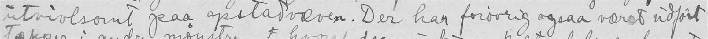utvivlsomt paa opstadvæven. Der har forøvrig ogsaa været udført
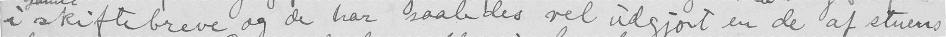i skiftebreve og de har saaledes vel udgjort en de af stuens
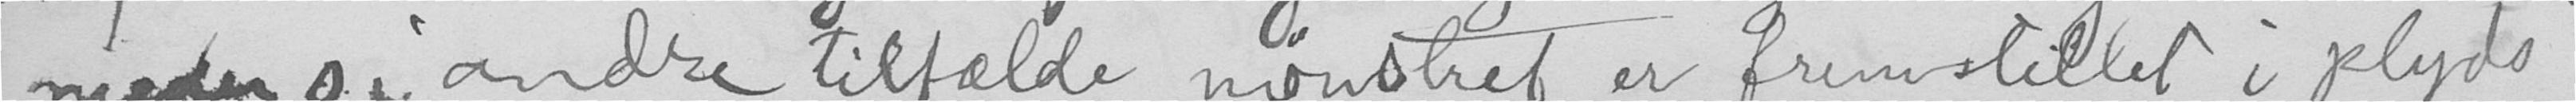medens andre tilfælde mønstret er fremstillet i plyds
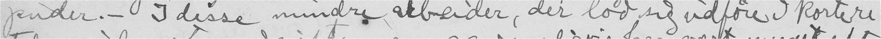puder. - I disse mindre arbeider, der lod sig udføre i kortere
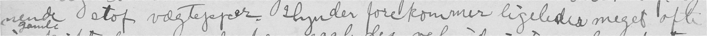nende stof vægtepper Hynder forekommer ligeledes meget ofte
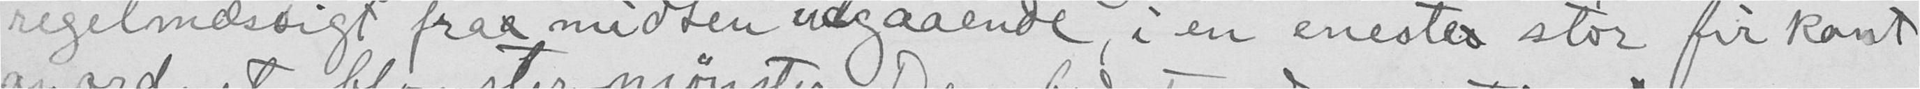regelmessigt fra  midten udgaaende i en eneste stor fin kant
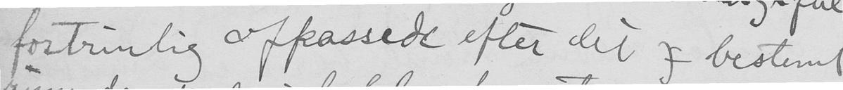fortrulig afpassede efter dei i bestemt
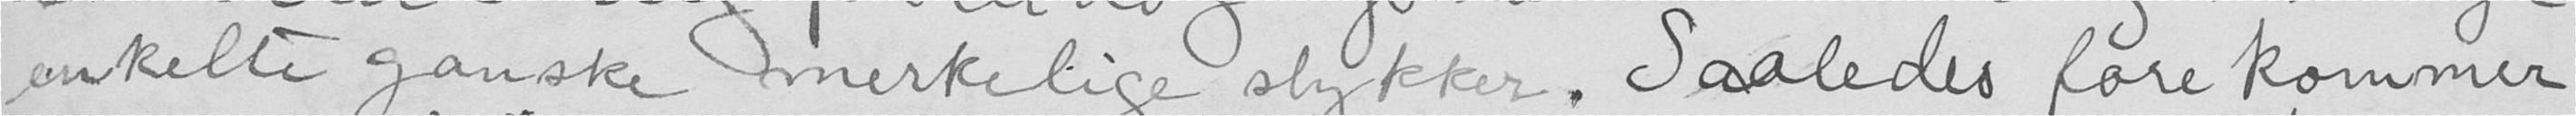enkelte ganske merkelige stykker. Saaledes forekommer
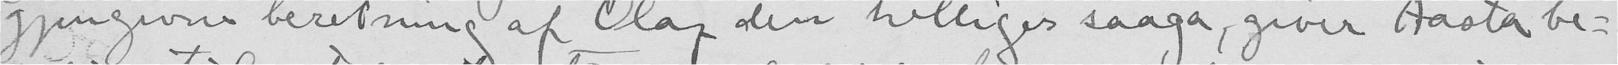gjengivne beredning af Olaf den helliges saaga giver Aasta be-
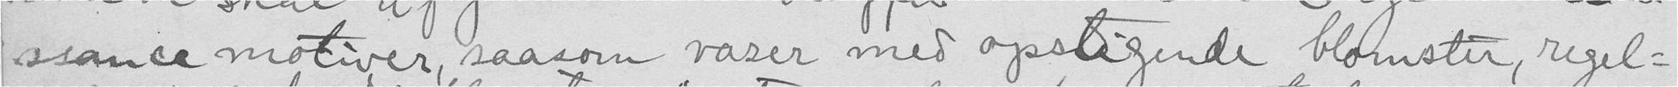sance motiver saasom vaser med opstigende blomster, regel-
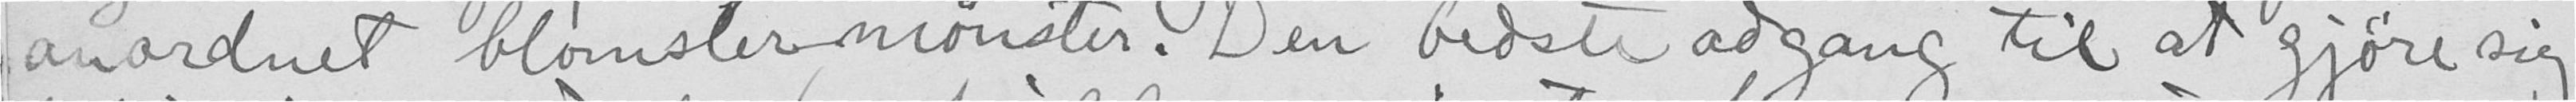anordnet blomstermønster. Den bedste adgang til at gjøre sig
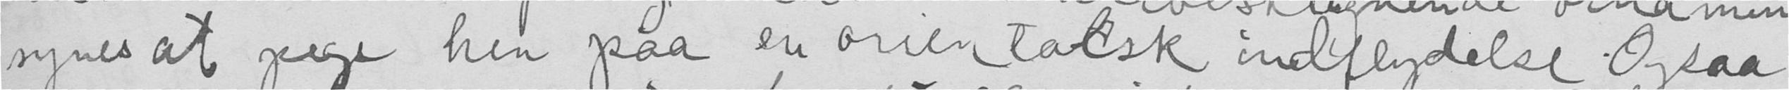synes at pege hen paa en orientalsk indflydelse. Ogsaa
